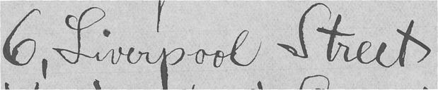6, Liverpool Street
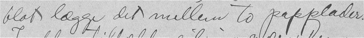blot lægge det mellem to papplader.
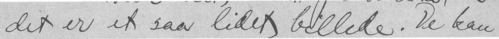det er et saa lidet billede. De kan
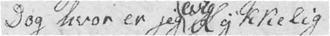Dog hvor er jeg evig Lykkelig
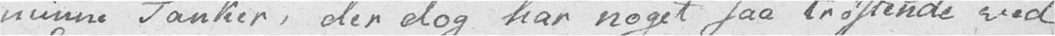minne Tanker, der dog har noget saa trøstende ved
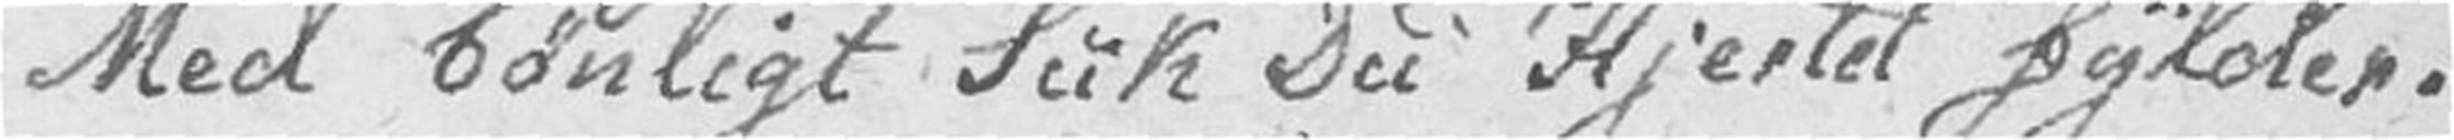Med bønligt Suk Du Hjertet fylder.
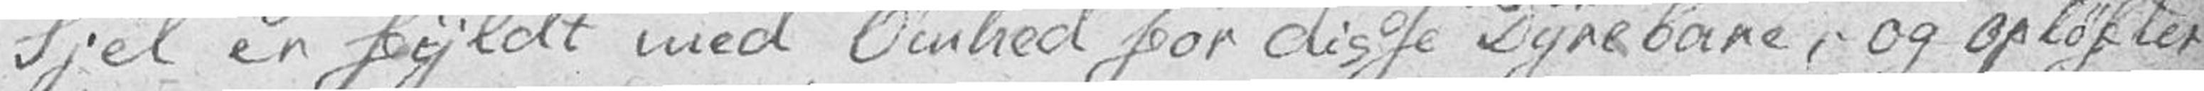Sjel er fyldt med Ømhed for disse Dyrebare, og opløfter
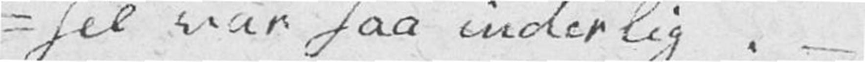-sel var saa inderlig. –
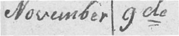November 9de
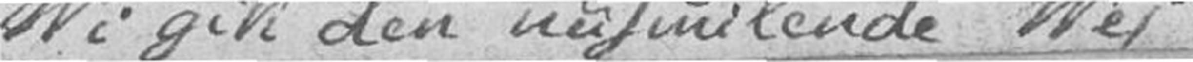Vi gik den nu smilende Vej
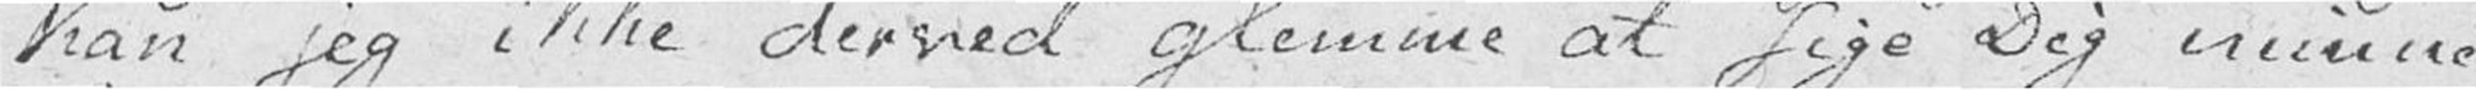kan jeg ikke derved glemme at sige Dig minne
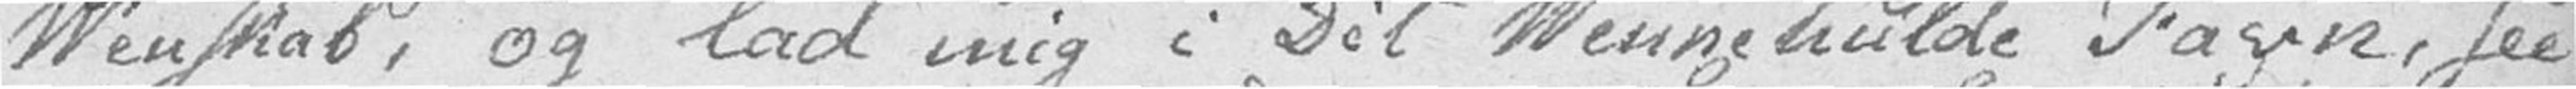Venskab, og lad mig i Dit Vennehulde Favn, see
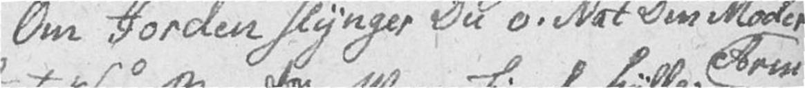Om Jorden slynger Du o! Nat Din Moder Arm
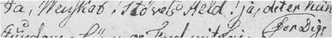Ja, Venskab! Støvets Held! ja, det er kun for Dig,
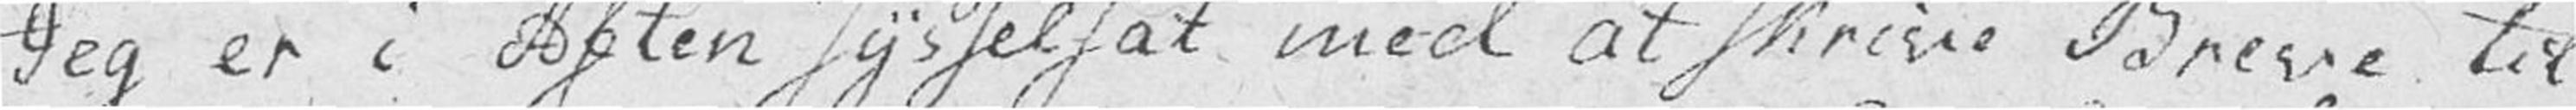Jeg er i Aften sysselsat med at skrive Breve til
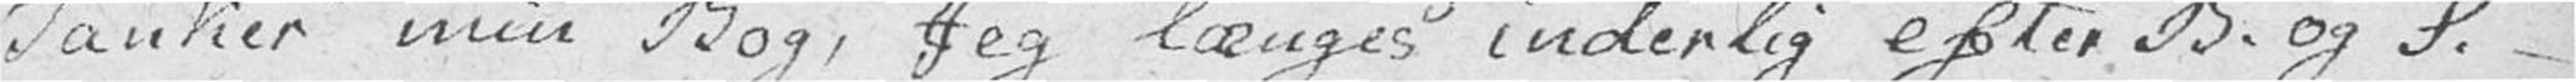Tanker min Bog, Jeg længes inderlig efter B. og S. –
